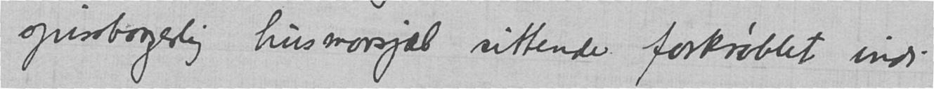spissborgerlig husmorsjæl sittende forkrøblet indi
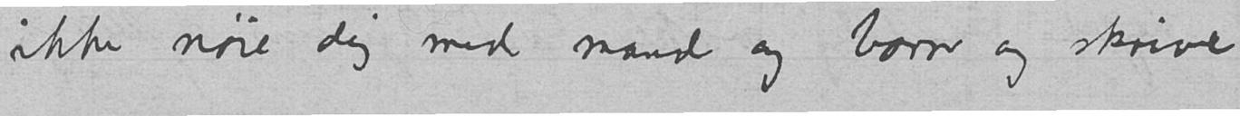ikke nøie dig med mand og barn og skrive
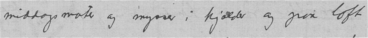middagsmater og mysser i kjælder og paa loft
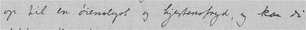op til en øienslyst og hjertensfryd, og kan du
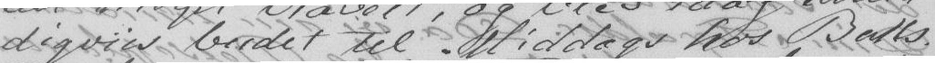digviis budet til Middag hos Bulls.
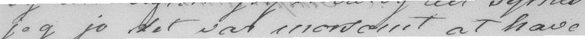jeg jo det var morsomt at have
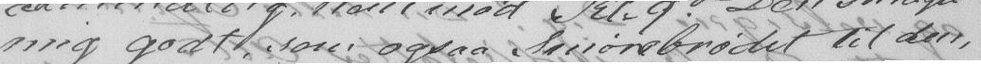mig godt som ogsaa Smørebrødet til den,
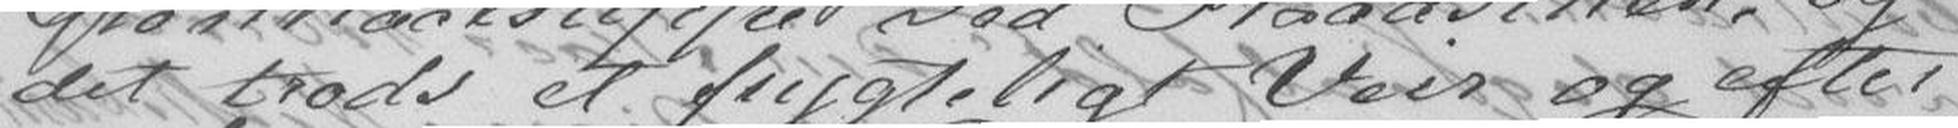det trods et frygteligt Veir og efter
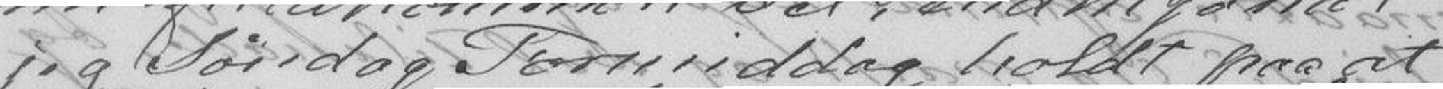jeg Søndag Formiddag holdt paa at
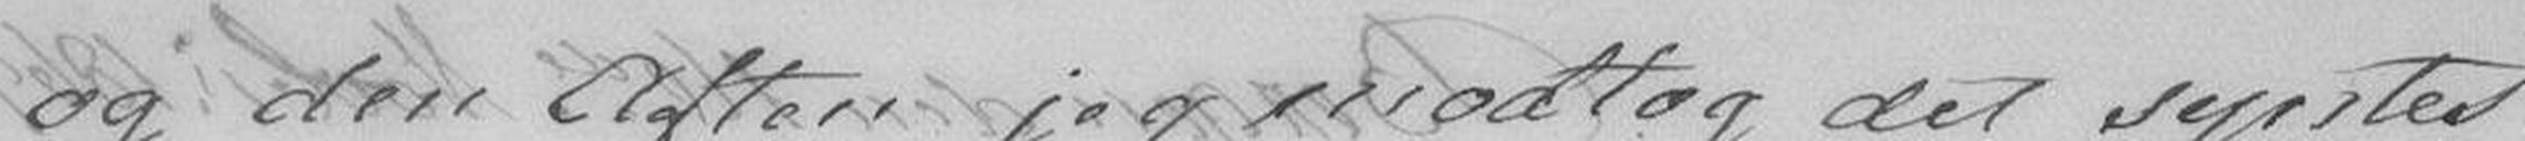og den Aften jeg modtog det syntes
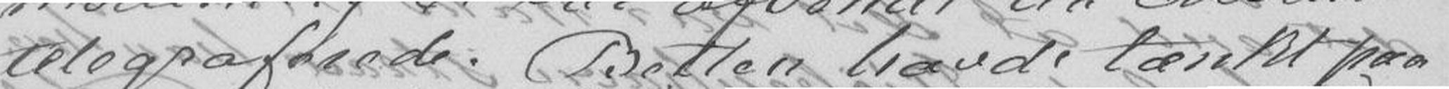telegraferede. Betten havde tænkt paa
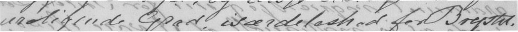uroligende Grad. isærdeleshed for Brystet.
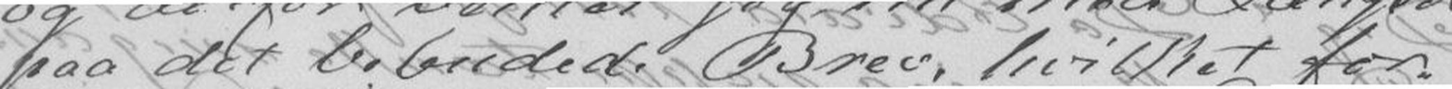paa det bebuded Brev, hvilket for-
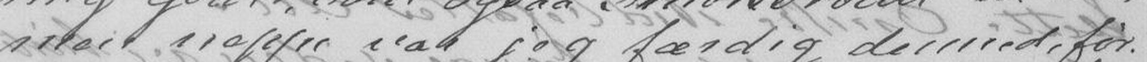men neppe var jeg færdig dermed, før
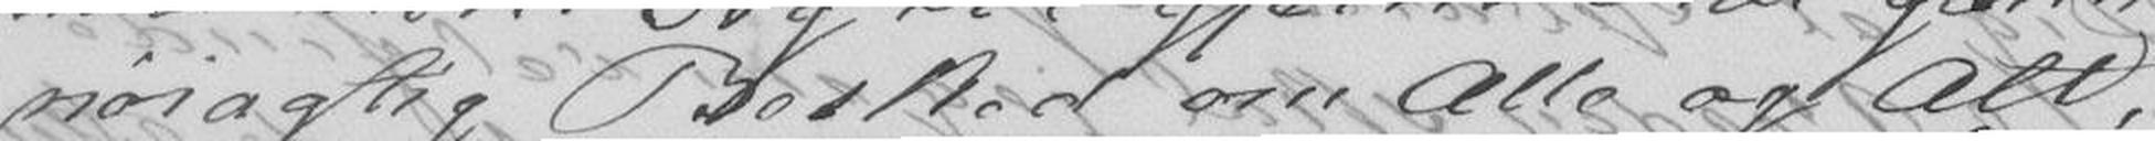nøiagtig Besked om Alle og Alt,
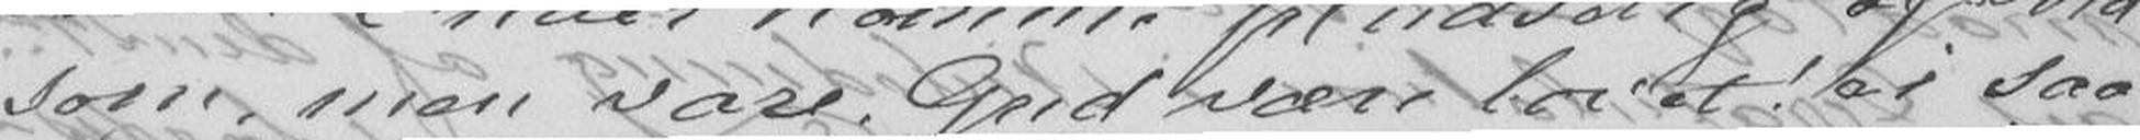som, men vare, Gud være lovet! ei saa
